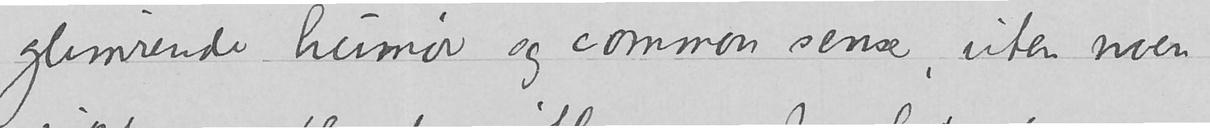glimrende humør og common sense, uten noen
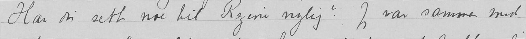Har du sett noe til Regine nylig? Jeg var sammen med
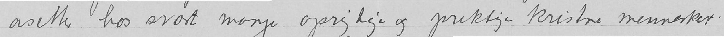avsetter hos svært mange oprigtige og prektige kristne mennesker.
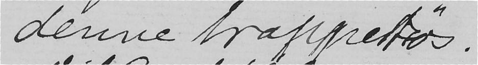denne trappetøs.
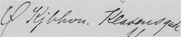Ø Kjbhvn. Klasensgade
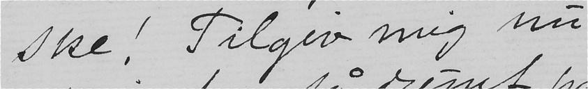ske! Tilgiv mig nu
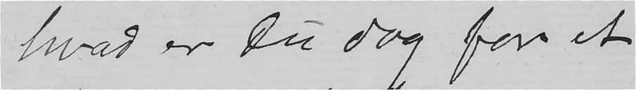hvad er Du dog for et
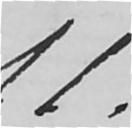11.
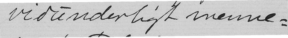vidunderligt menne-
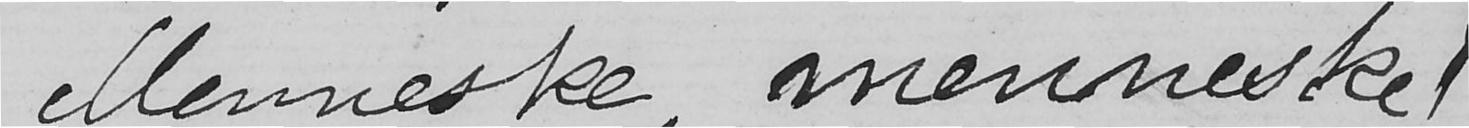Menneske, menneske!
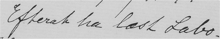Efterat ha læst Labo-
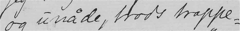og unåde, trods trappe-
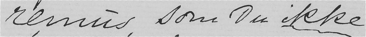remus, som Du ikke
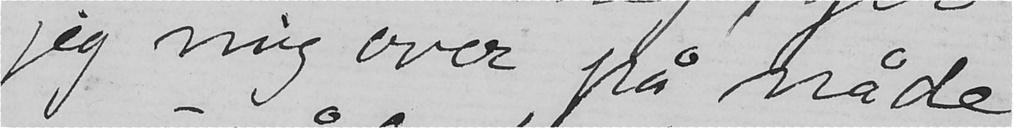jeg mig over på nåde
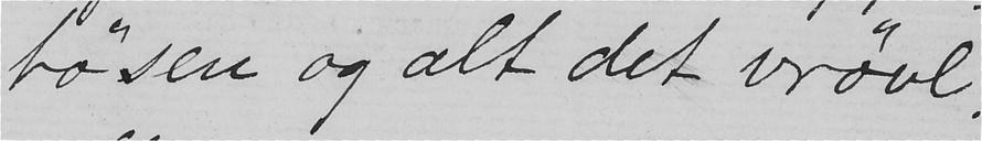tøsen og alt det vrøvl.
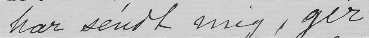har sendt mig, gir
